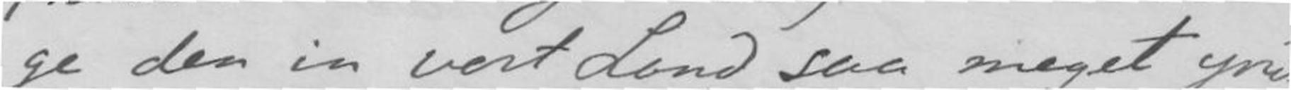ge den in vert land saa meget ynde
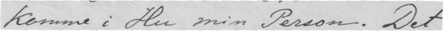komme i Hu min Person. Det
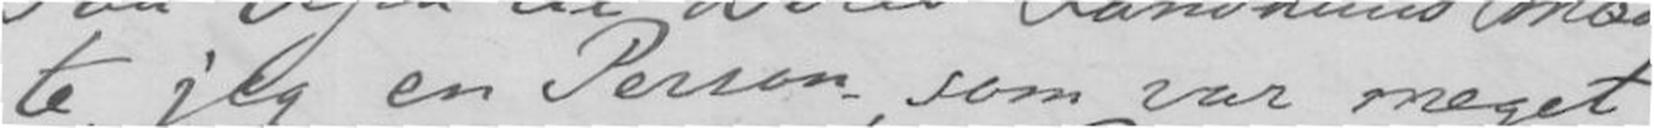te jeg en Person som var meget
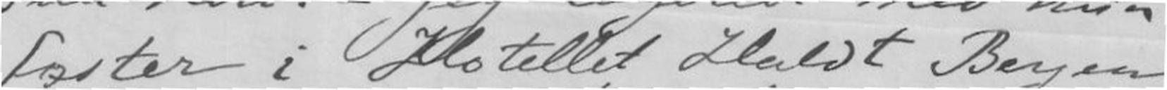Søster i Hotellet Holdt Bergen
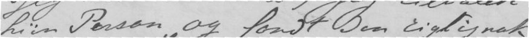hiin Person og fandt den rigtignok
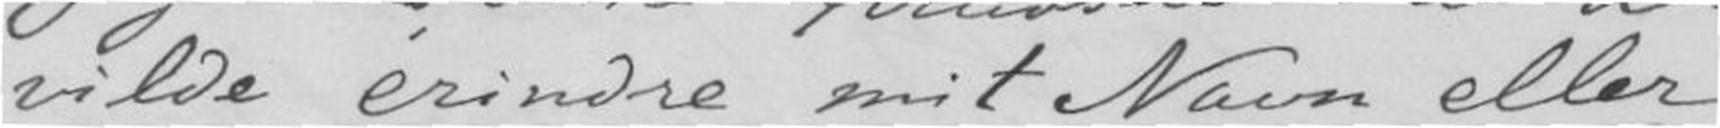vilde érindre mit Navn eller
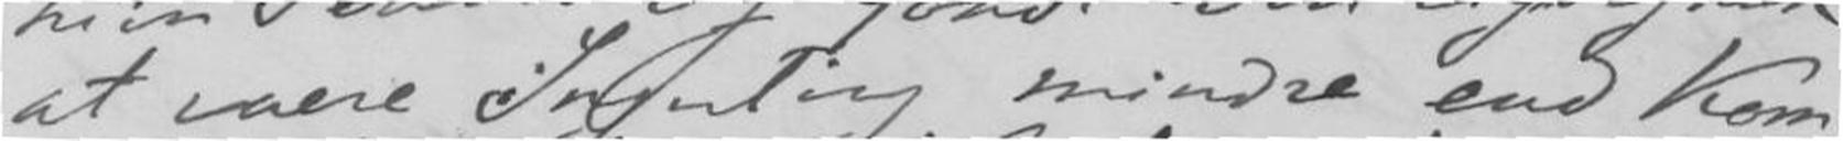at være Ingenting mindre en Kom-
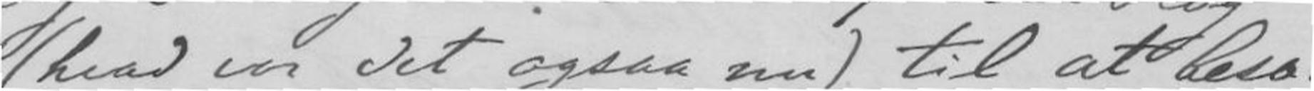(hvad var det ogsaa nu). til at besø-
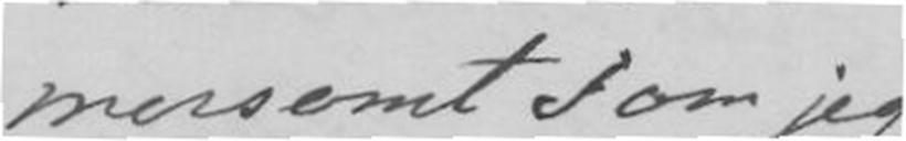morsomt som jeg
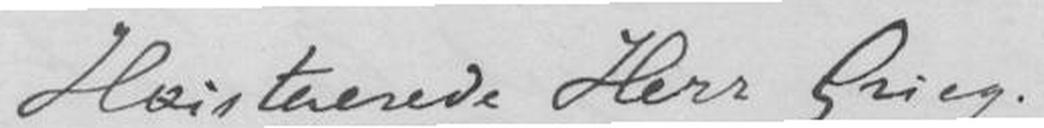Høistærede Herr Grieg.
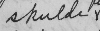skulde
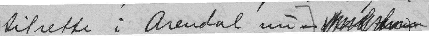tilrette i Arendal nu -
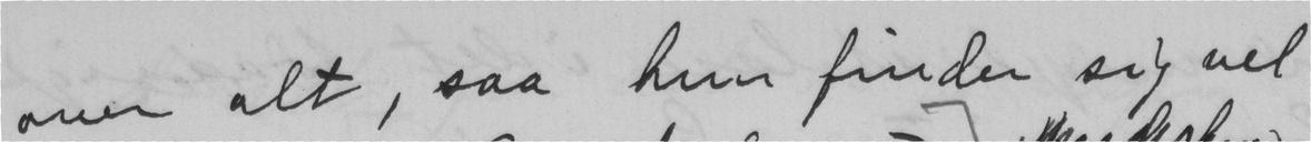over alt, saa hun finder sig vel
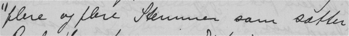"flere og flere Stemmer som sætter
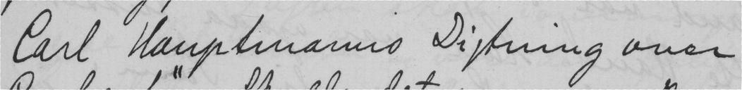Carl Hauptmanns Digtning over
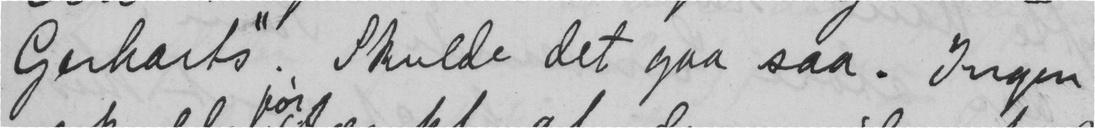Gerharts". Skulde det gaa saa. Ingen
